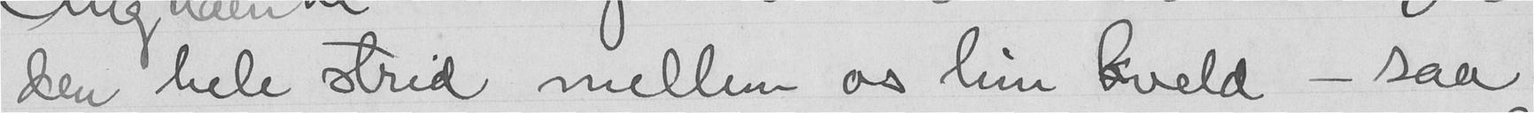den hele strid mellem os hin kveld, - saa
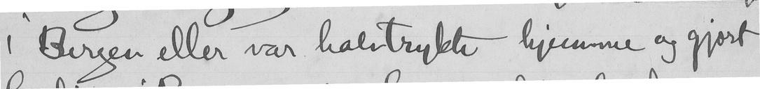i Bergen eller var halvtrykte hjemme og gjort
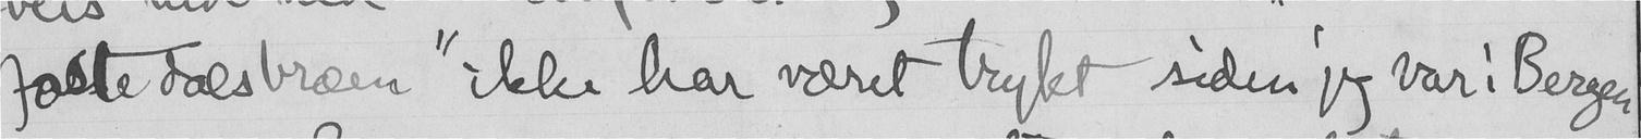Jostedalsbræen" ikke har været trykt siden jeg var i Bergen.
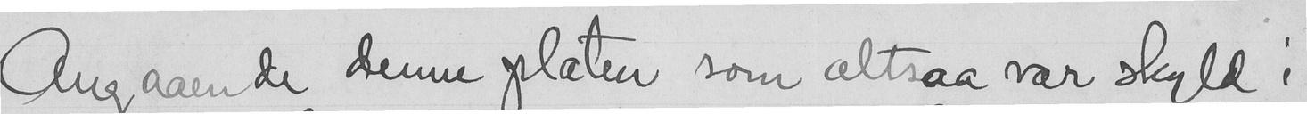Angaaende denne platen som altsaa var skyld i
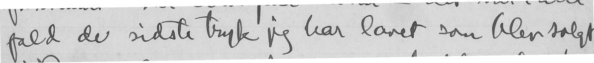fald de sidste tryk jeg har lavet som blev solgt
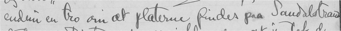endnu en tro om at platerne findes paa Sandalstrand
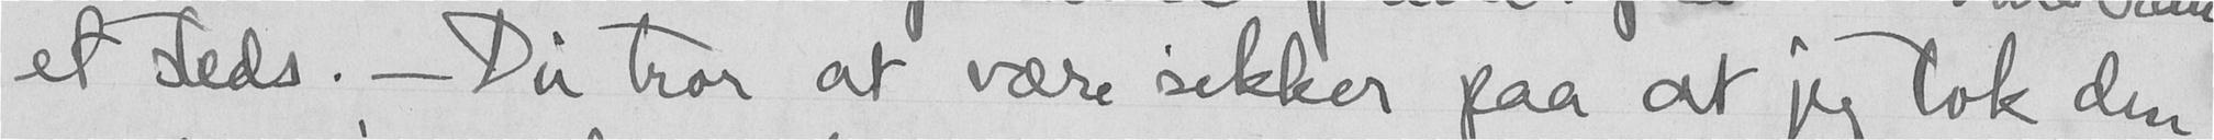et steds. - Du tror at være sikker paa at jeg tok den
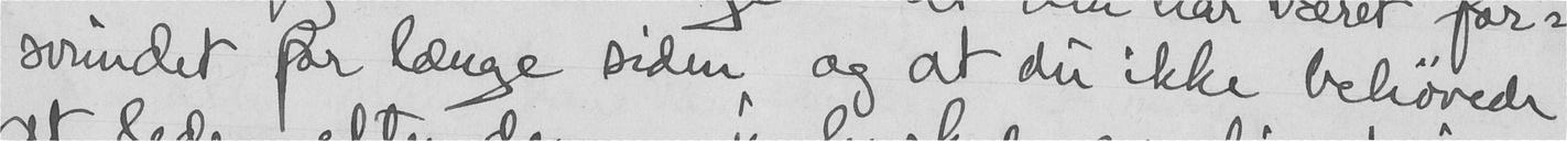svundet for længe siden os at du ikke behøvede
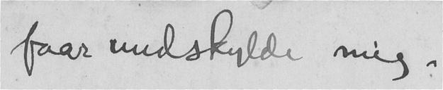faar undskylde mig.
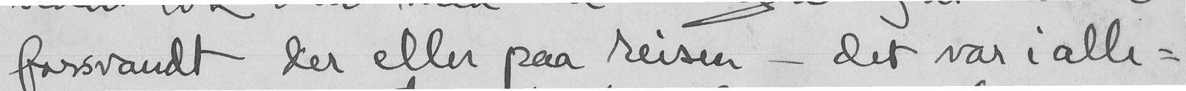forsvandt der eller paa reisen - det var i alle-
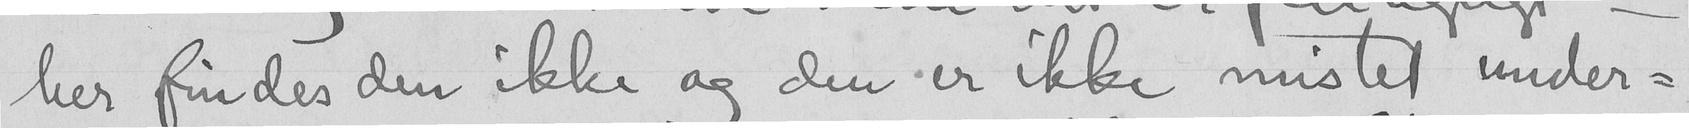her findes den ikke og den er ikke mistet under-
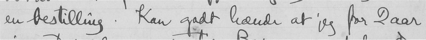en bestilling. Kan godt hænde at jeg for 2 aar
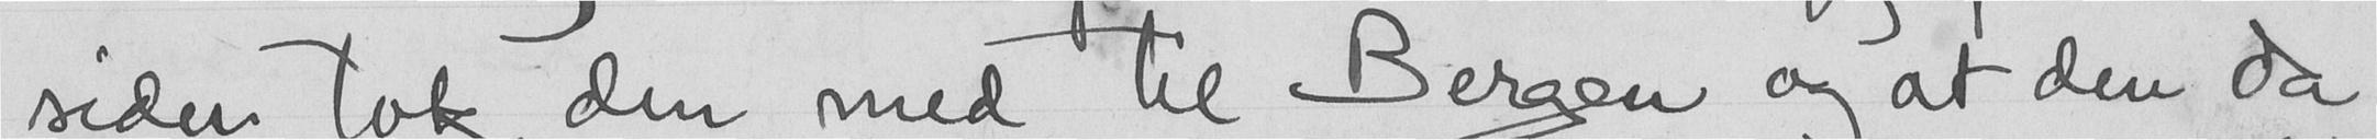siden tok den med til Bergen og at den da
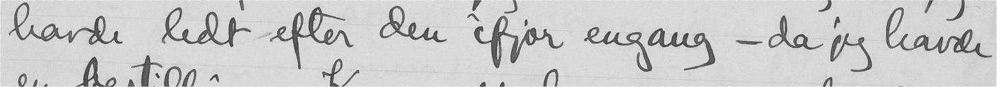havde ledt efter den ifjor engang - da jeg havde
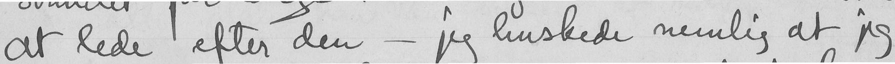at lede efter den - jeg huskede nemlig at jeg
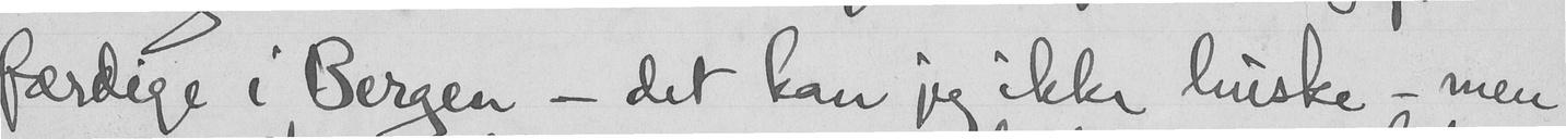færdige i Bergen, - det kan jeg ikke huske - men
